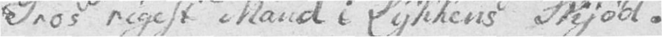Tros rigest Mand i Lykkens Skjød.
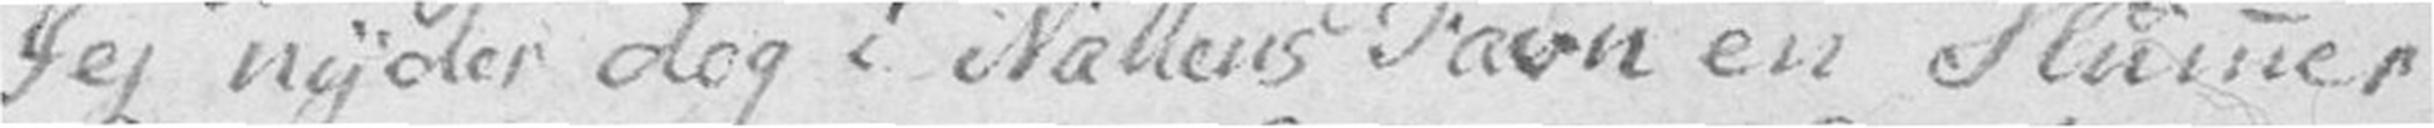Jeg nyder dog i Nattens Favn en Slummer
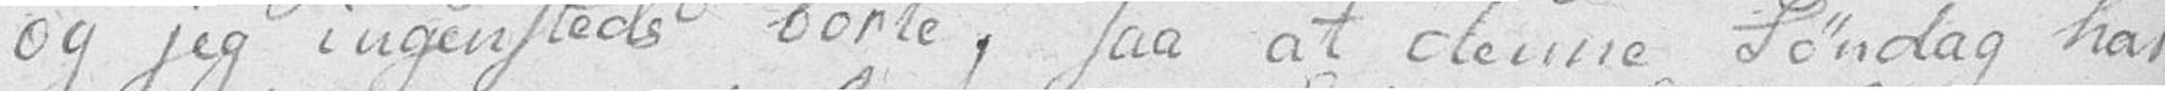og jeg ingensteds borte, saa at denne Søndag har
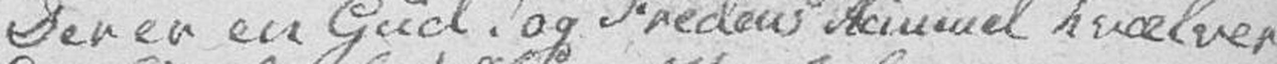Der er en Gud! og Fredens Himmel hvælver
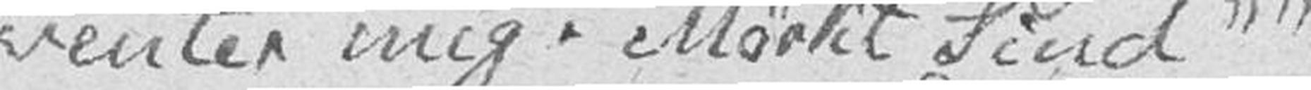venter mig. Mørkt Sind ""
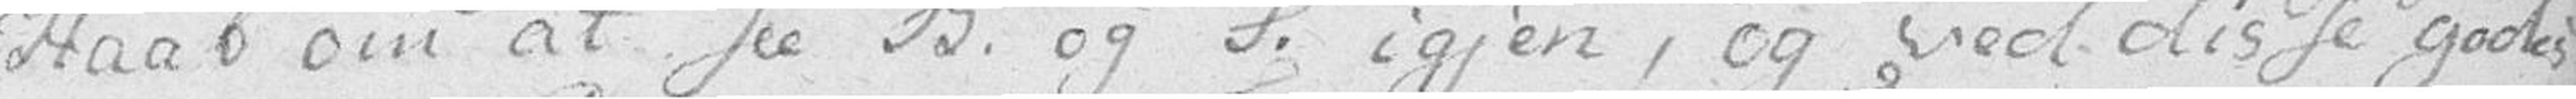Haab om at see B. og S. igjen, og ved disse godes
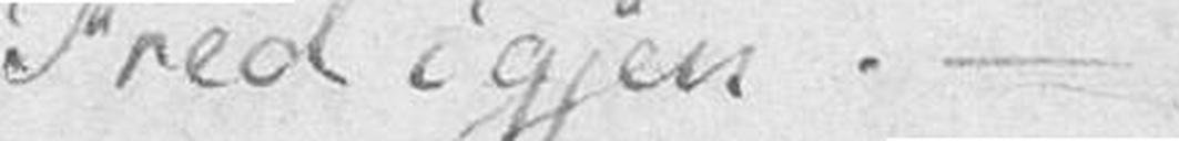Fred igjen. –
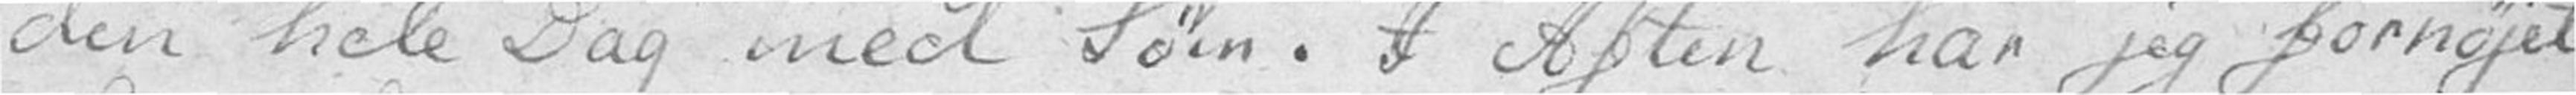den hele Dag med Søm. I Aften har jeg fornøjet
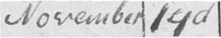November 14d
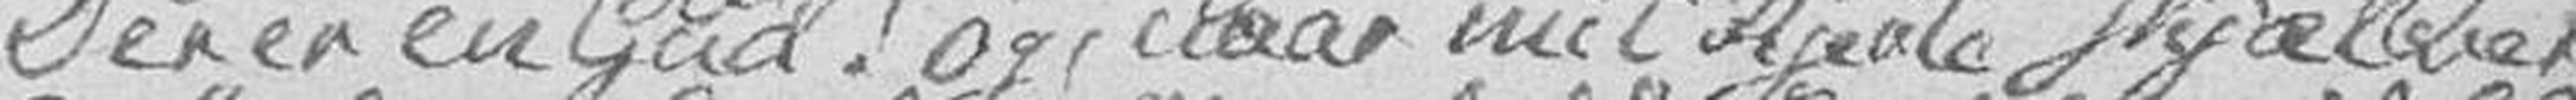Der er en Gud! og, naar mit Hjerte skjælver
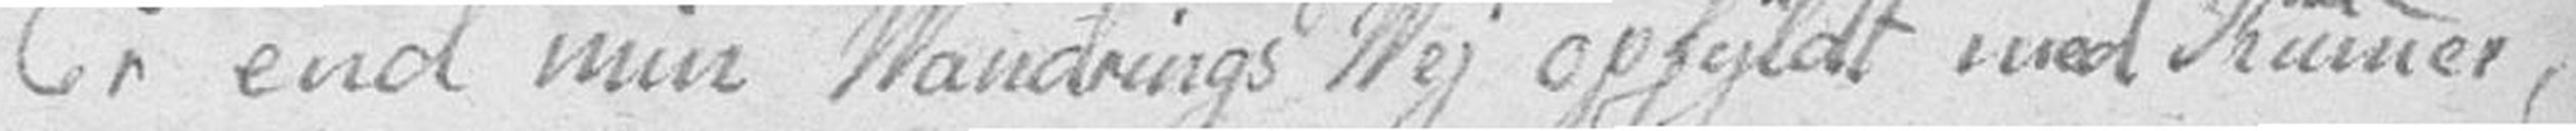Er end min Vandrings Vej opfyldt med Kummer,
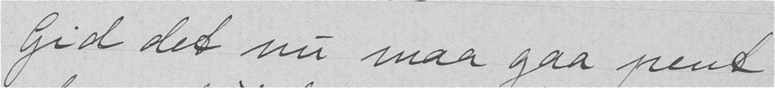Gid det nu maa gaa pent
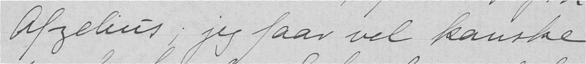Afzelius; jeg faar vel kanske
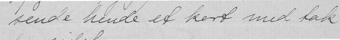sende hende et kort med tak
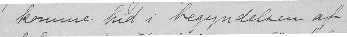komme hid i begyndelsen af
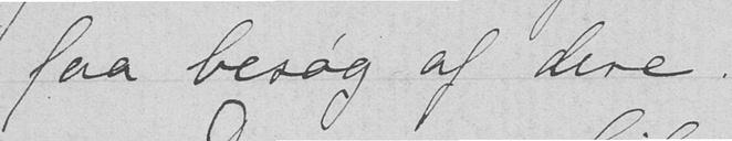faa besøg af dere!
